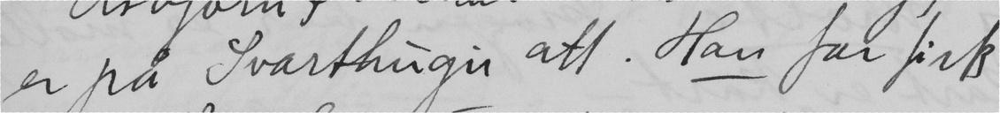er på Svarthugir att. Han far fisk
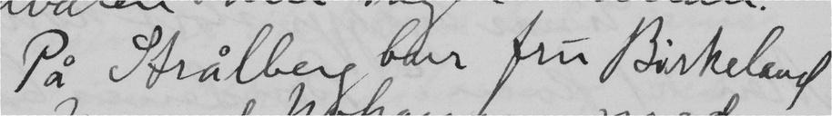På Strålberg bur Fru Birkeland
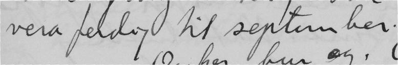vera ferdig til september.
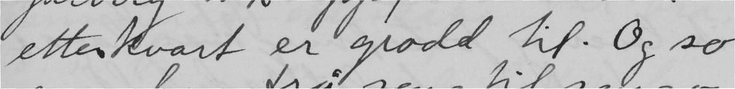etterkvart er grodd til. Og so
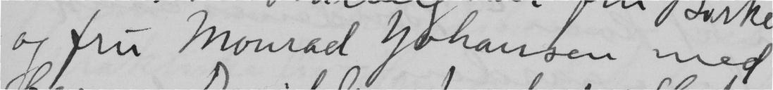og fru Monrad Johansen med
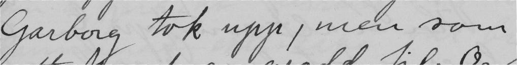Garborg tok upp; men som
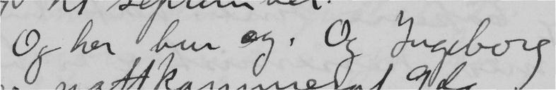Og her bur eg. Og Ingeborg
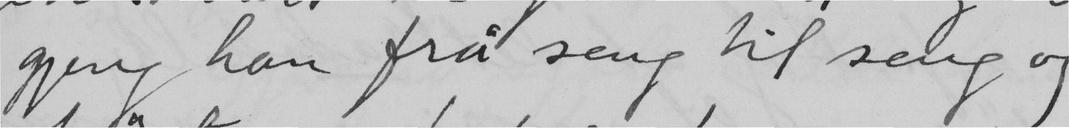gjeng han frå seng til seng og
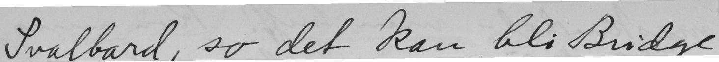Svalbard so det kan bli Bridge -
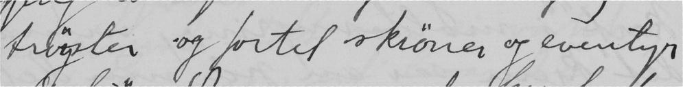tröyster og fortel skröner og eventyr -
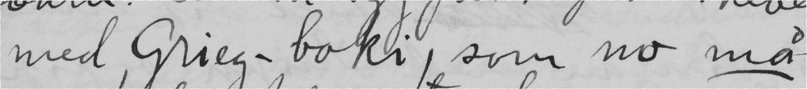med Grieg-boki, som no må
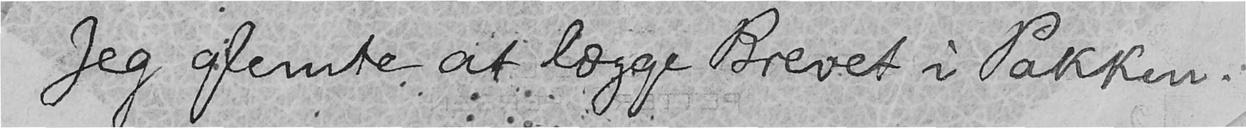Jeg glemte at lægge Brevet i Pakken.
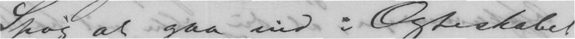Spøg at gaa ind i Ægteskabet
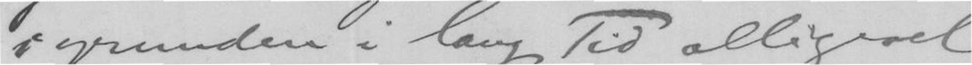igrunden i lang Tid alligevel
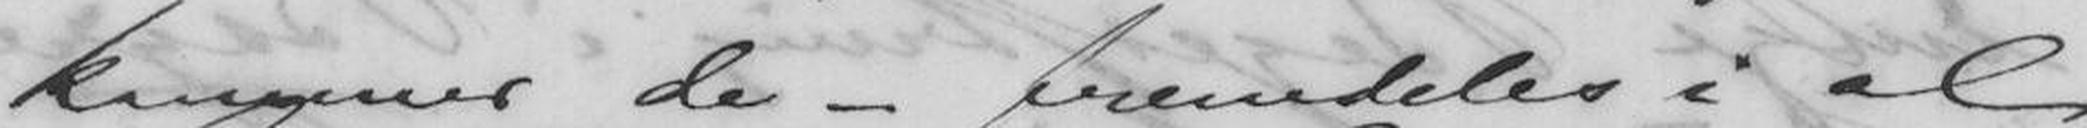kommer de - fremdeles i al
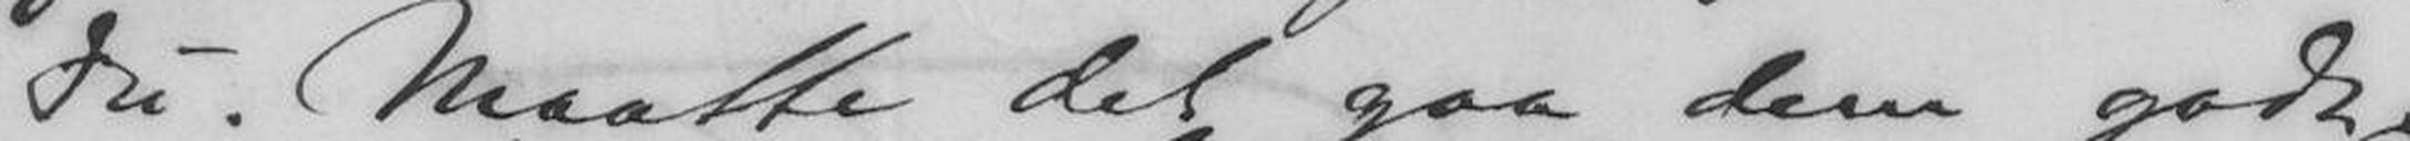Du. Maatte det gaa dem godt.
